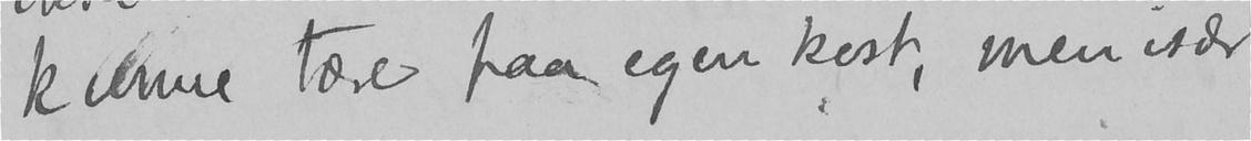kunne tære paa egen kost, men især
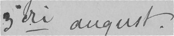3die august.
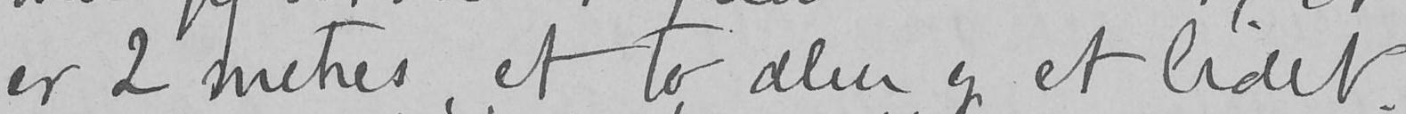er 2 metres, et to alen og et lidet.
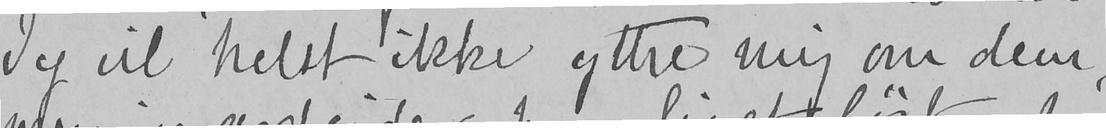Jeg vil helst ikke yttre mig om dem,
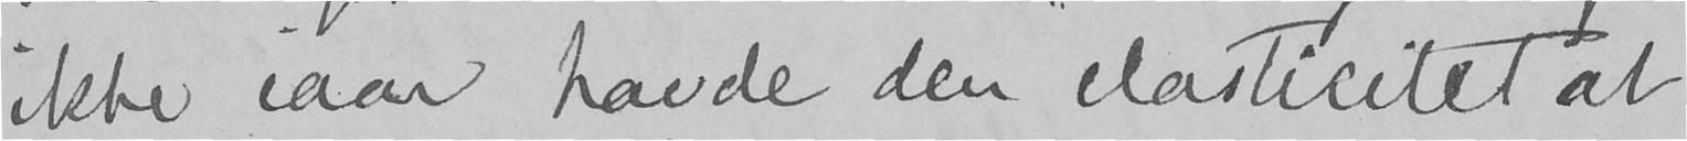ikke iaar havde den elasticitet at
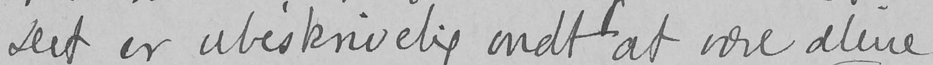Det er ubeskrivelig ondt at være alene
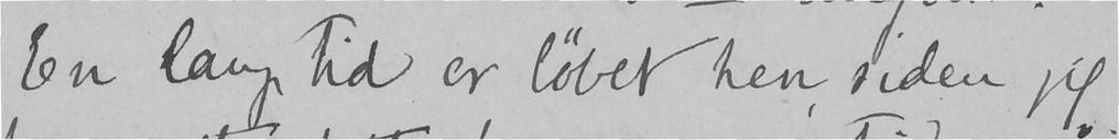En lang tid er løbet hen, siden jeg
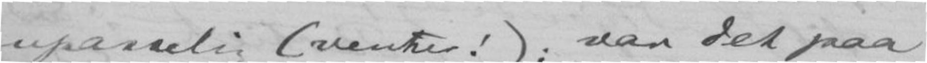upasselig (venter!), var det paa
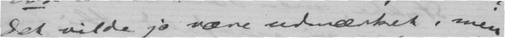det vilde jo være udmærket, men
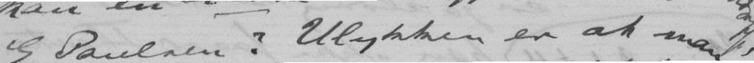E Paulsen? Ulykken er at man
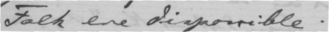Folk ere disponible.
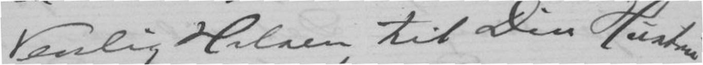Venlig Hilsen til Din Hustru
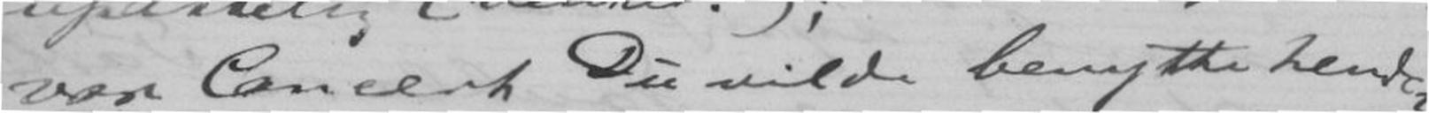vor Concert Du vilde benytte hende?
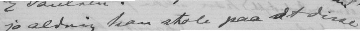jo aldrig kan stole paa at disse
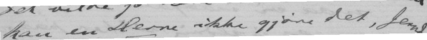kan en Herre ikke gjøre det, Jerndoff,
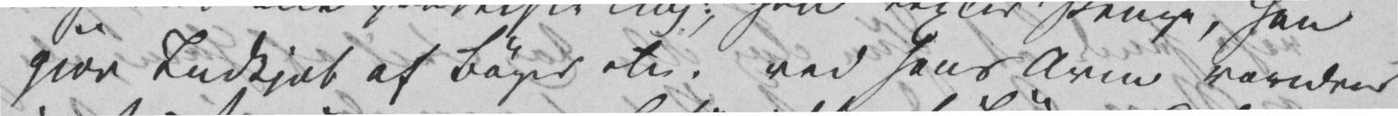gjør Indkjøb af Bøger etc. ved hans Arm vandrer
